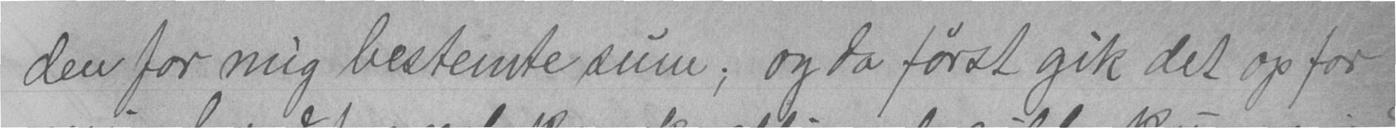den for mig bestemte sum; og da først gik det op for
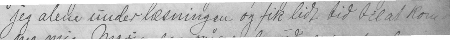jeg alene under læsningen og fik lidt tid til at kom-
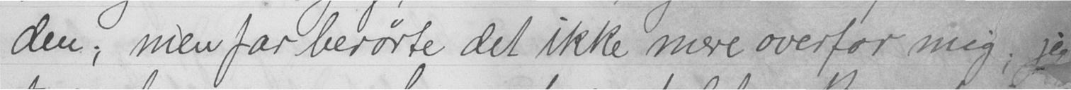den; men far berørte det ikke mere overfor mig; jeg
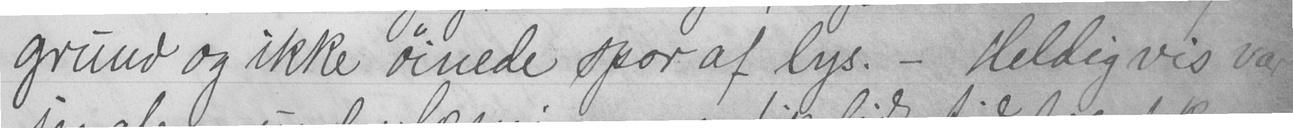grund og ikke øinede spor af lys. - Heldigvis var
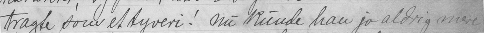tragte som et tyveri! nu kunde han jo aldrig mere
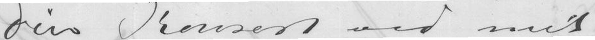Din Konsert ved mit
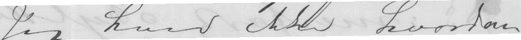Jeg hved ikke hvordan
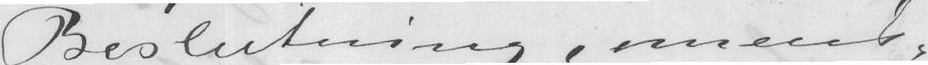Beslutning, omend
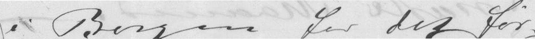i Bergen for det før-
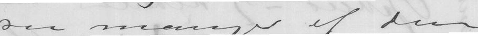saa mange af den
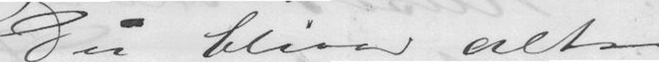Du bliver altsaa
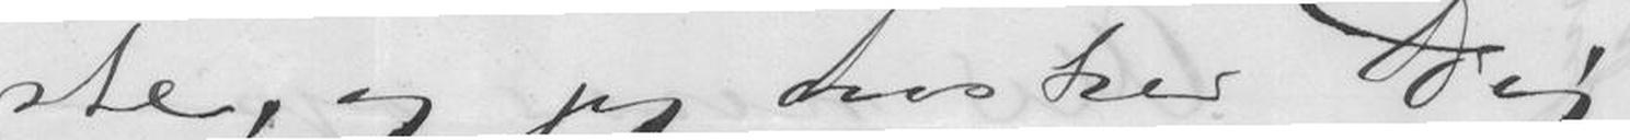ste, og jeg ønsker Dig
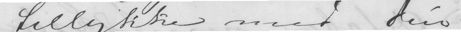tillykke med Din
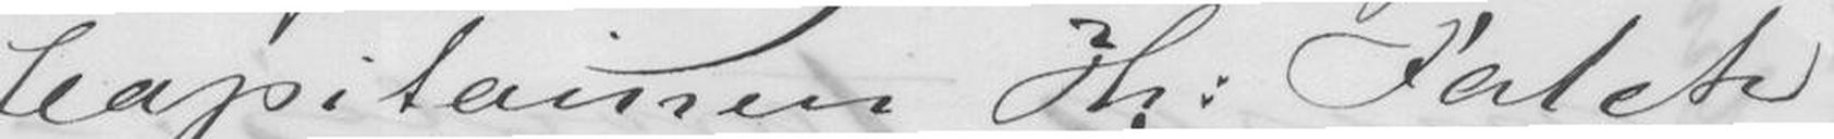Capitainen Hr: Falck
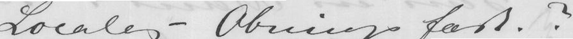Locales - Obnings fæst?
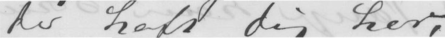de haft Dig her,
